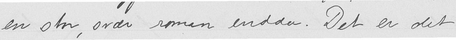en stor, svær roman endda. Det er det
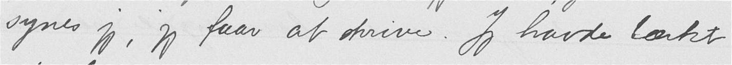synes jeg, jeg faar at skrive. Jeg havde tænkt
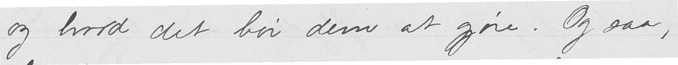og hvad det bør dem at gjøre. Og saa,
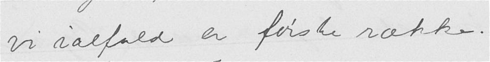vi ialfald er første række.
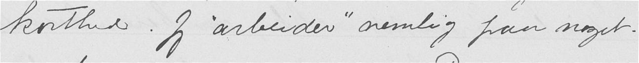korthed. Jeg "arbeider" nemlig paa noget,
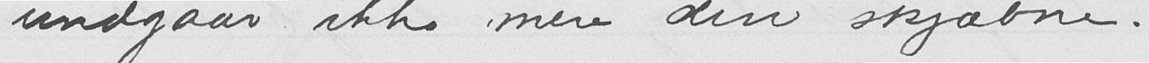undgaar ikke mere din skjæbne.
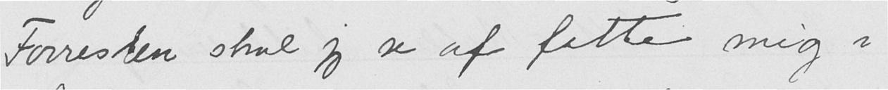Forresten skal jeg se at fatte mig i
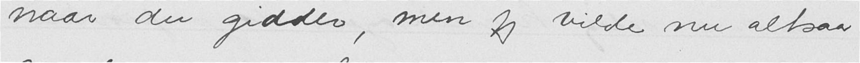naar du gidder, men jeg vilde nu altsaa
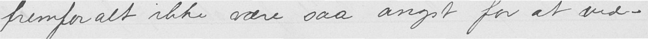fremforalt ikke være saa angst for at ved-
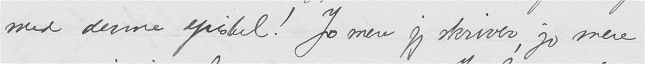med denne epistel! Jo mere jeg skriver, jo mere
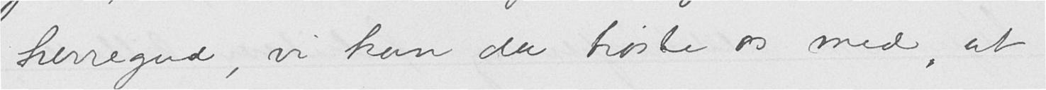herregud, vi kan da trøste os med, at
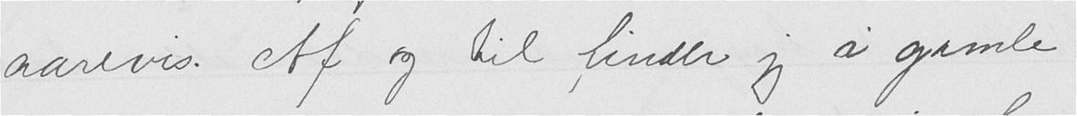aarevis. Af og til finder jeg i gamle
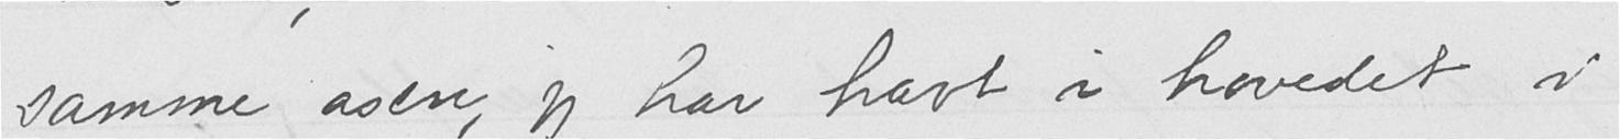samme asen, jeg har havt i hovedet i
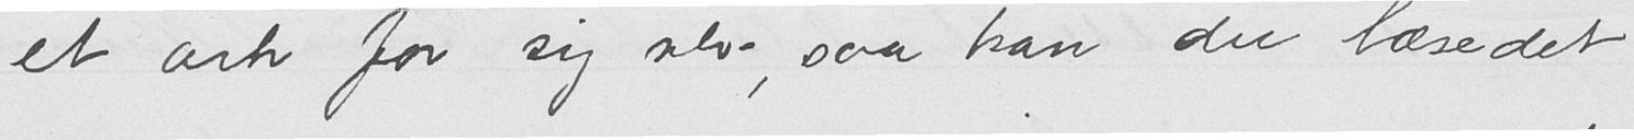et ark for sig selv, saa kan du læse det
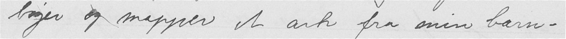bøger og mapper et ark fra min barn-
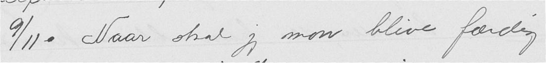9/11. Naar skal jeg mon blive færdig
